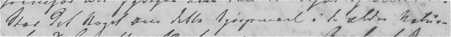Stod det Noget om dette Spørgsmaal i de ældre Natur-
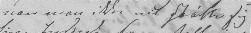naar man ikke vil støtte sig
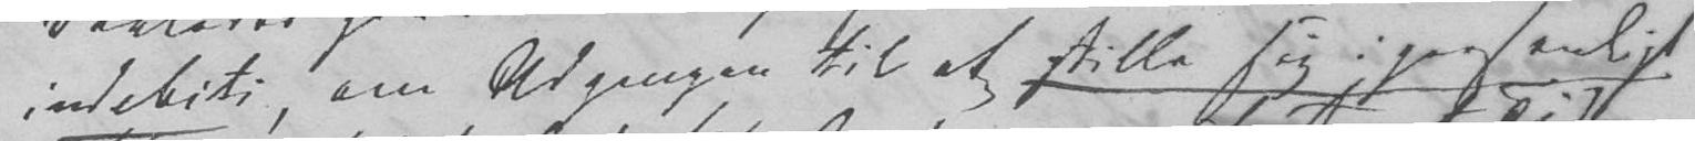indebiti, om Adgangen til at stille sig i personligt
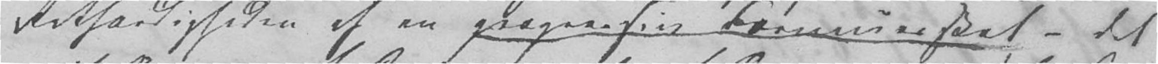Retfærdigheden af en progressiv Formuesskat - det
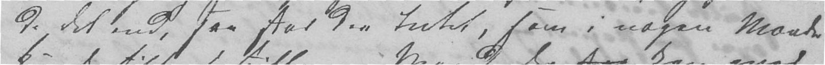de det end, saa stod der Intet, som i nogen Maade
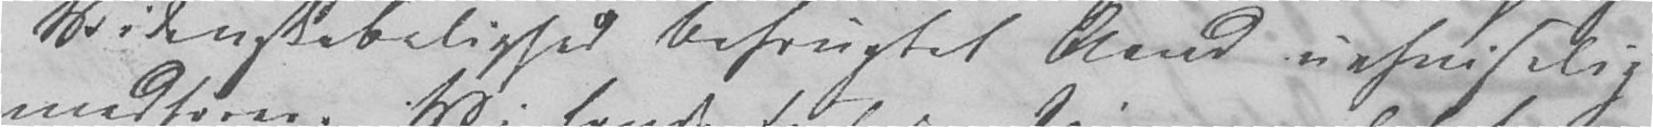Videnskabelighet befrugtet Aand uafviselig
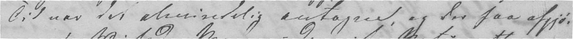Tid var det almindelig antagne, og der faa afgjø-
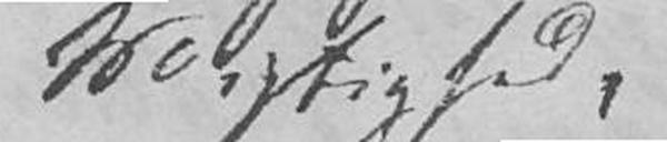Vigtighed,
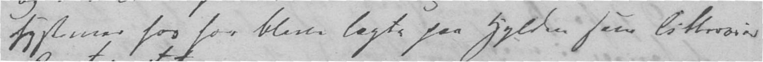systemer hos hos bleve lagte paa Hylden som litteraire
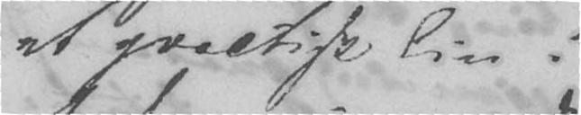et practisk Liv i
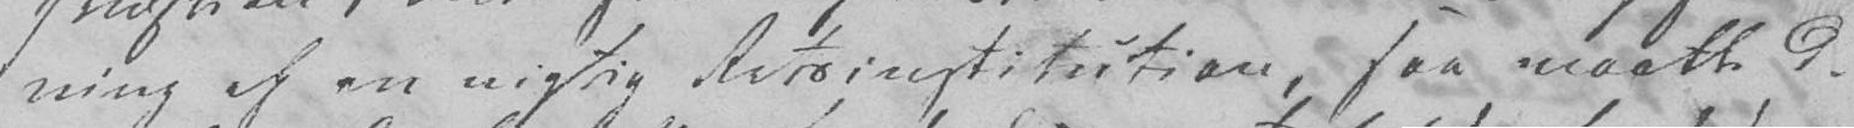ning af en vigtig Retsinstitution, saa maatte de
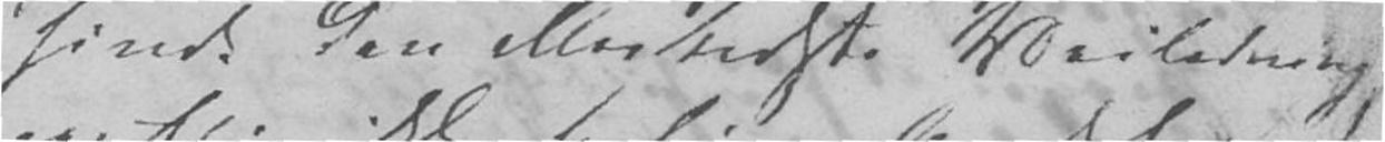finde den allerbedste Veiledning
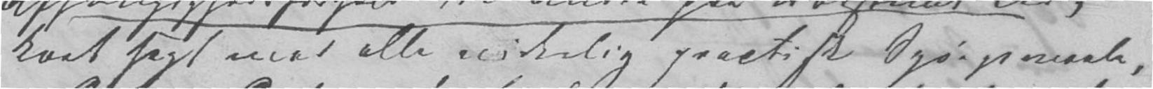kort sagt med alle virkelig practiske Spørgsmaale,
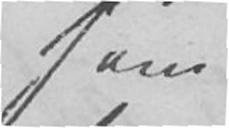som
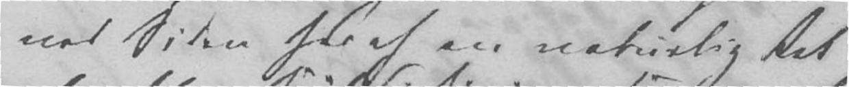ved Siden heraf en naturlig Ret
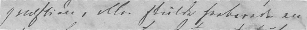qvæstion, eller skulde forberede en
